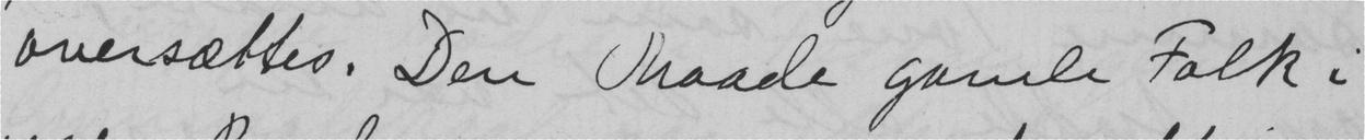oversættes. Den Maade gamle Folk i
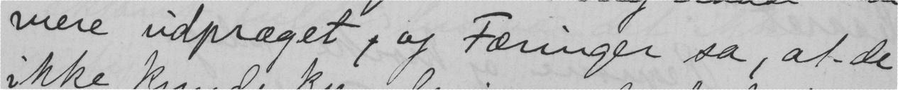mere udpræget, og Færinger sa, at de
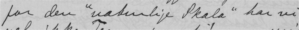for den "naturlige Skala" har vi
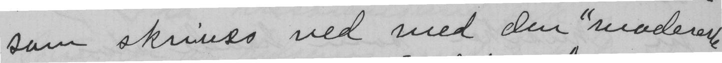som skrives ned med den "modererte
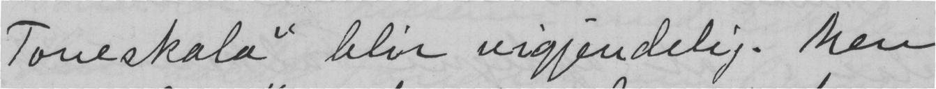Toneskala" blir uigjendelig. Men
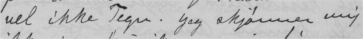vel ikke Tegn. Jeg skjønner mig
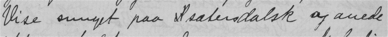Vise sunget paa  sætersdalsk og anede
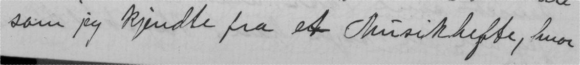som jeg kjendte fra et Musikhefte, hvor
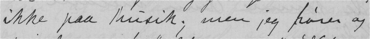ikke paa Musik; men jeg hører og
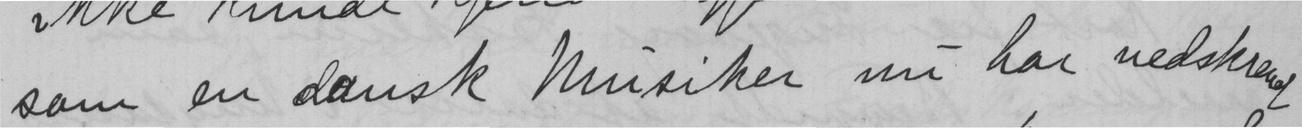som en dansk musiker nu har nedskrevet
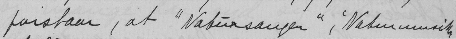forstaar, at "Natursangen", "Naturmusiken"
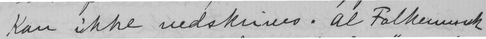kan ikke nedskrives. Al Folkemusik
